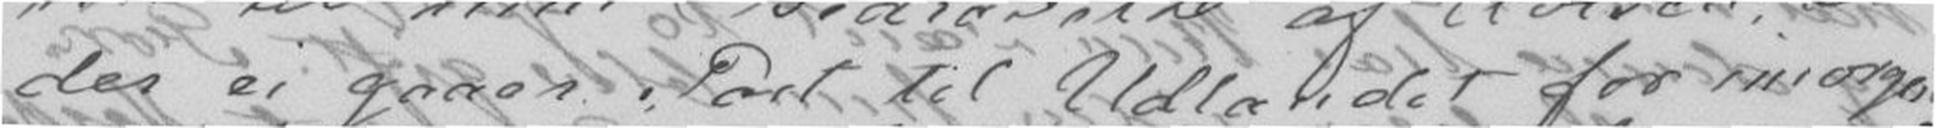der ei gaar Post til Udlandet for imorgen,
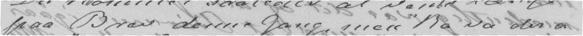paa Brev denne Gang, men ka va der a
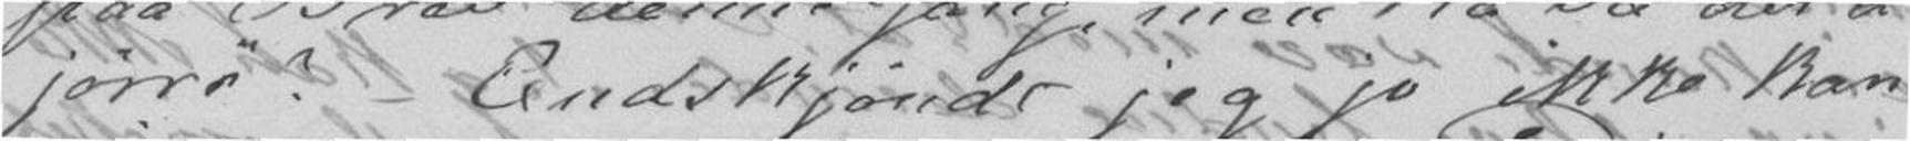jørrø? - Endskjøndt jeg jo ikke kan
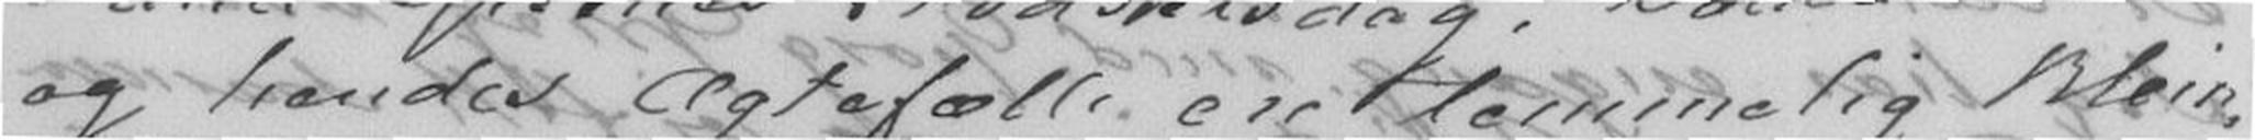og hendes Ægtefælle ere temmelig klein,
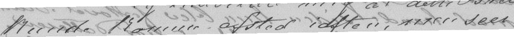kunde komme afsted iaften, men seer
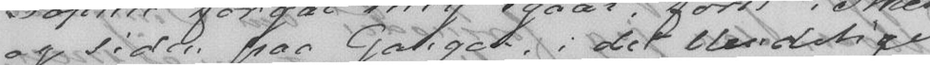og siden paa Gangen, i det Uendelige
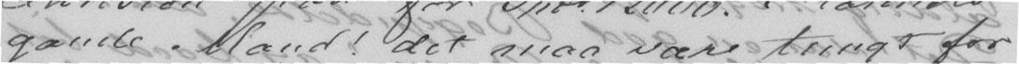gamle Mand! det maa være tungt for
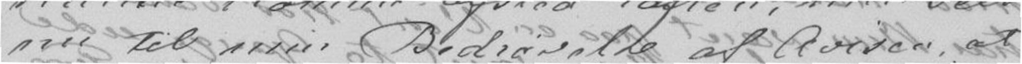nu til min bedrøvelse af Avisen, at
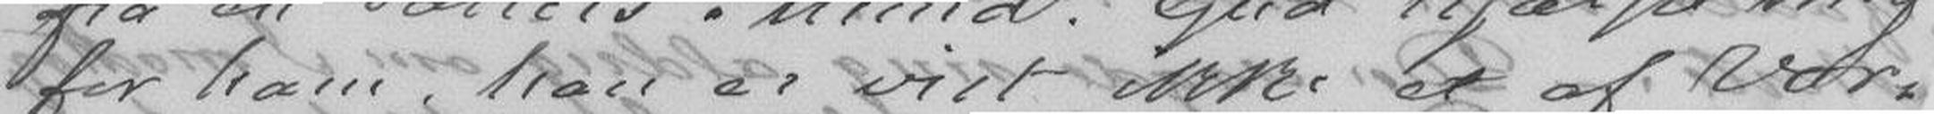for ham, han er vist ikke et af vore
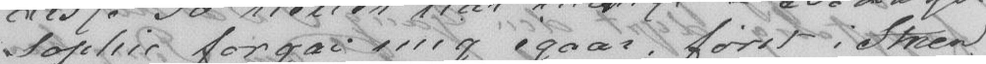Sophie forgav mig igaar, først i Stuen
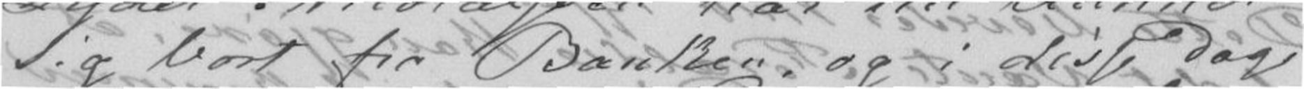sig bort fra Banken, og i disse Dage
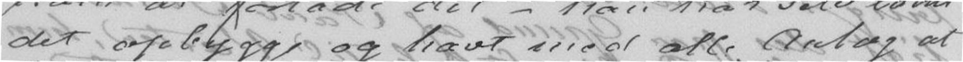det opbygge og havt med alle Anlæg at
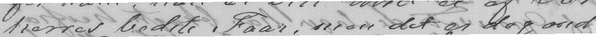herres bedste Faar, men der er dog ond
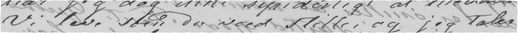Vi leve som Du veed stille, og jeg taler
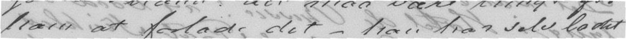ham at forlade det - han har selv ladet
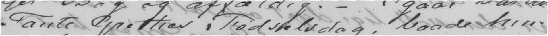Tante Grethes Fødselsdag, baade hun
